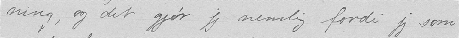ning, og det gjør jeg nemlig fordi jeg som
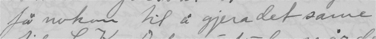få nokon til å gjera det same
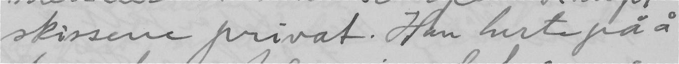skissene privat. Han lurte på å
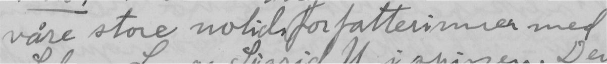våre store notidsforfatterinner med
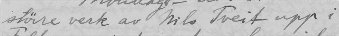större verk av Nils Tveit upp i
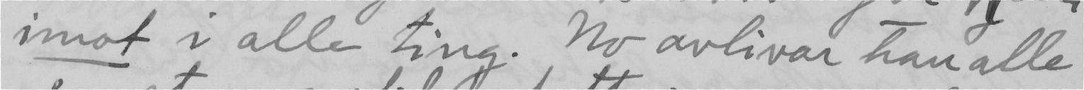imot i alle ting. No avlivar han alle
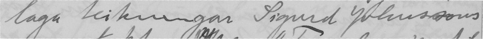laga teikningar Sigurd Johanssons
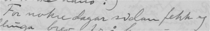For nokre dagar sidan fekk eg
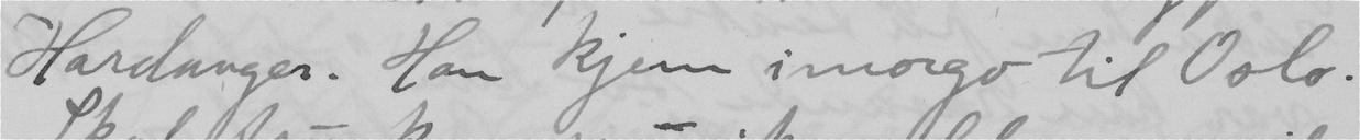Hardanger. Han kjem imorgo til Oslo.
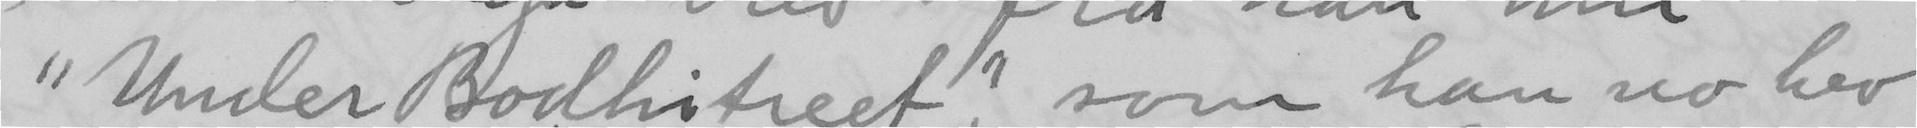"Under Bodhitreet" som han no hev
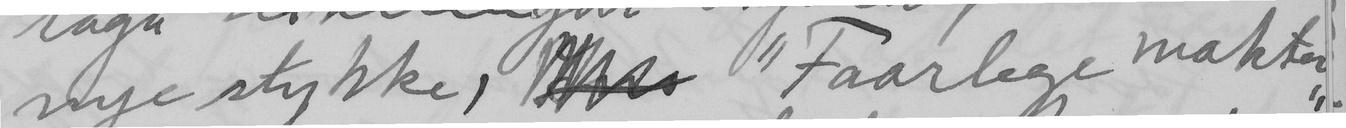nye stykke,  "Faarlege makter"
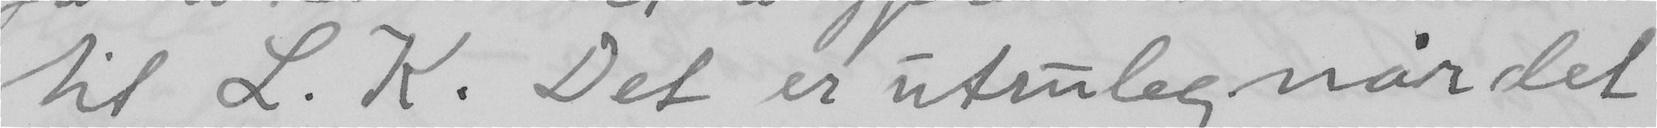til L. K. Det er utruleg når det
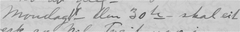Mondag - den 30te skal eit
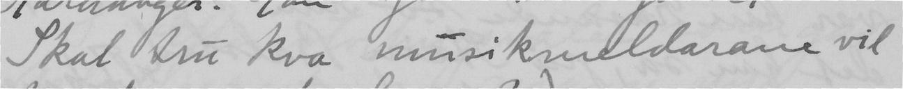Skal tru kva musikmeldarane vil
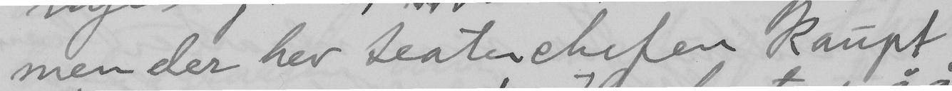men der hev teaterchefen kaupt
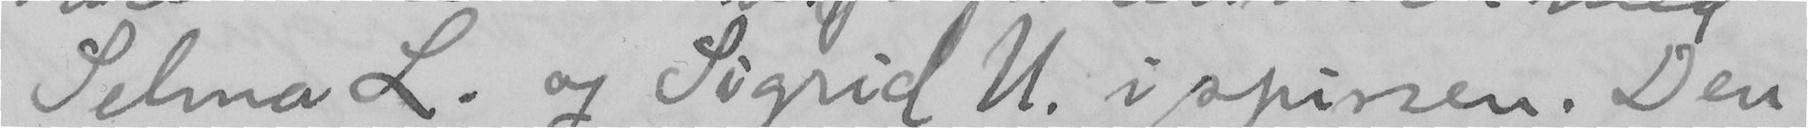Selma L. og Sigrid U. i spissen. Den
